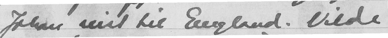Johan reist til England. Vilde
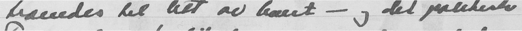troendes til litt av hvert - og det politiske
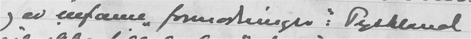og av infame "formodninger": Tyskland
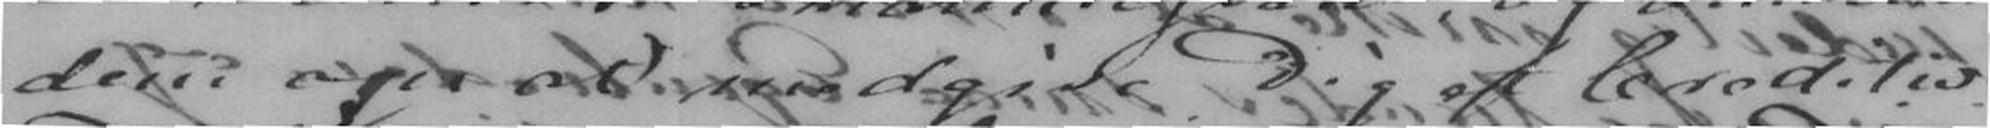dem om at medgive Dig et Creditiv.
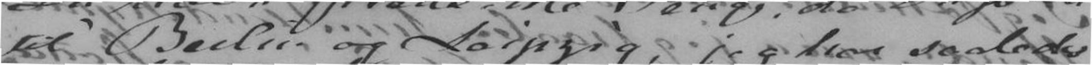til Berlin og Leipzig, jeg har saaledes
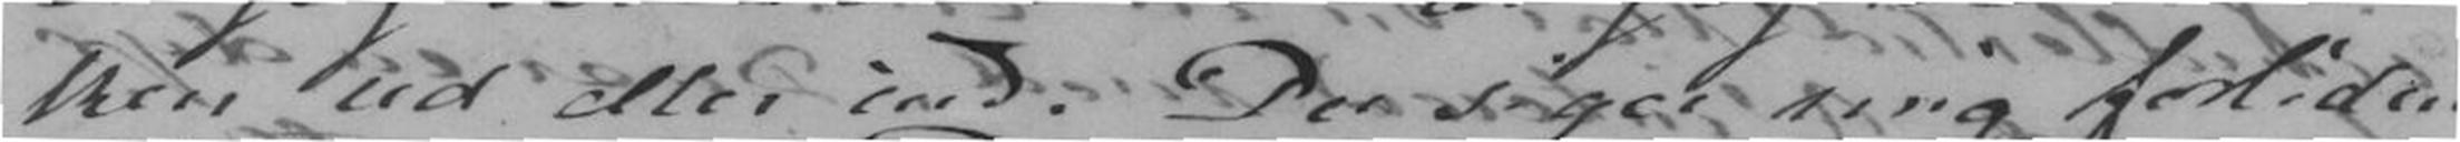ken ud eller ind. Du siger mig forliden
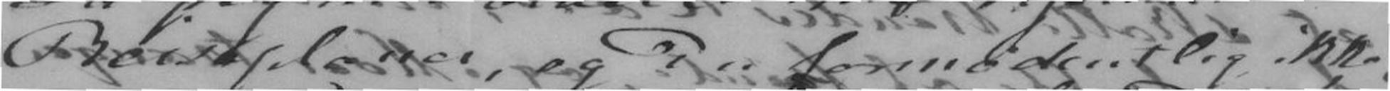Reiseplaner, og Du formodentlig ikke
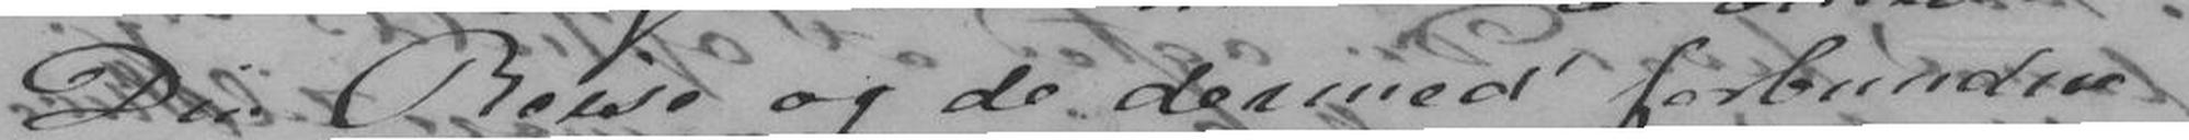Din Reise og de dermed forbundne
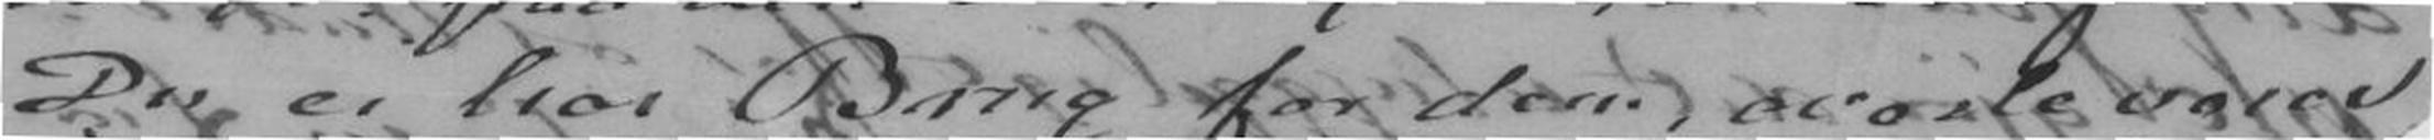Du ei har Brug for dem, overlever
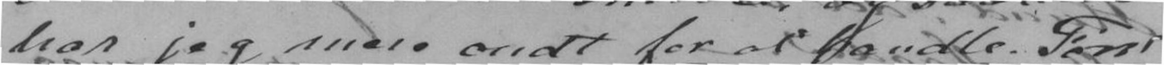har jeg mere ondt for at handle. Først
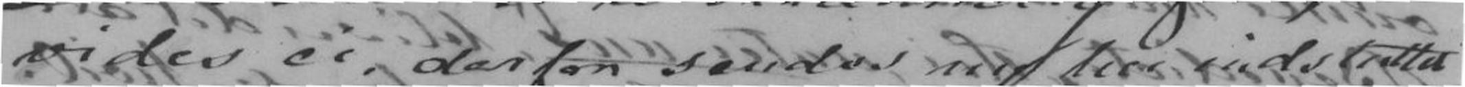vides ei derfor sender nu her indstrettet
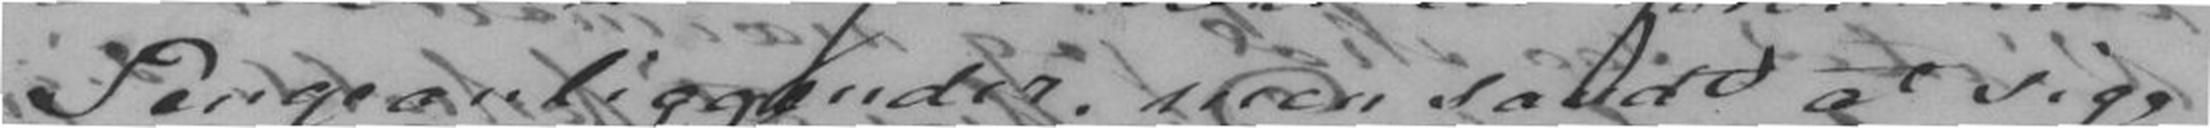Pengeanliggender, men sandt at sige
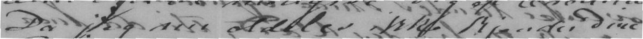Da jeg nu aldeles ikke kjender Dine
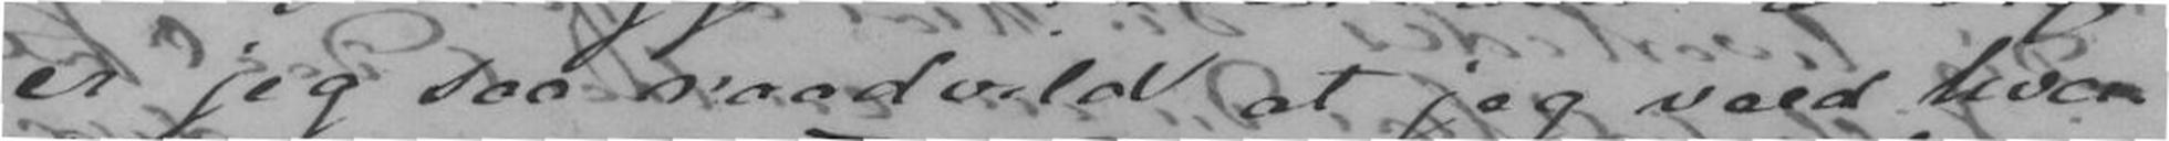er jeg saa raadvild at jeg veed hver-
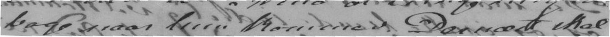bage naar hun kommer. Dernæst skal
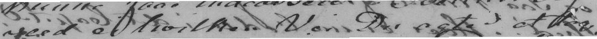veed ei hvilken Vei Du agter at tage,
